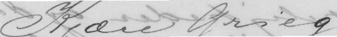Kjære Grieg
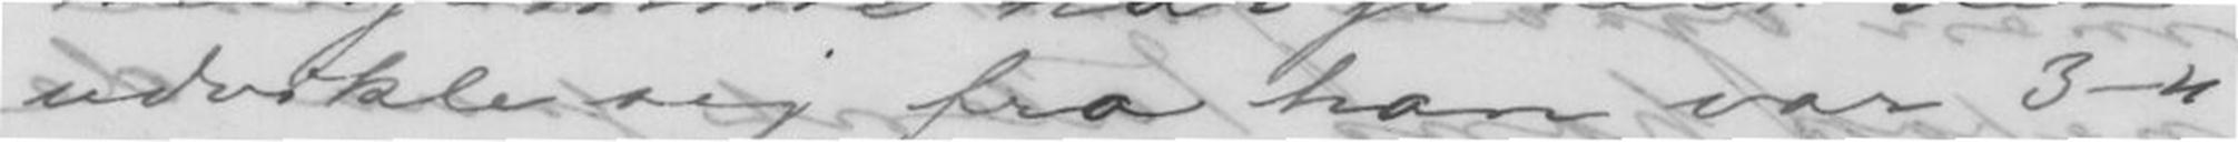udvikle sig fra han var 3-4
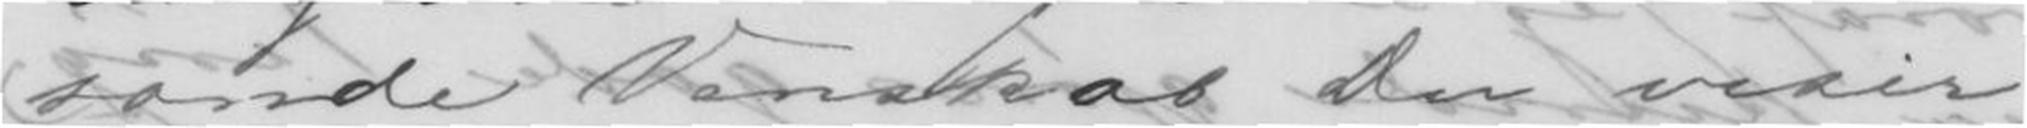sande Venskab Du viser
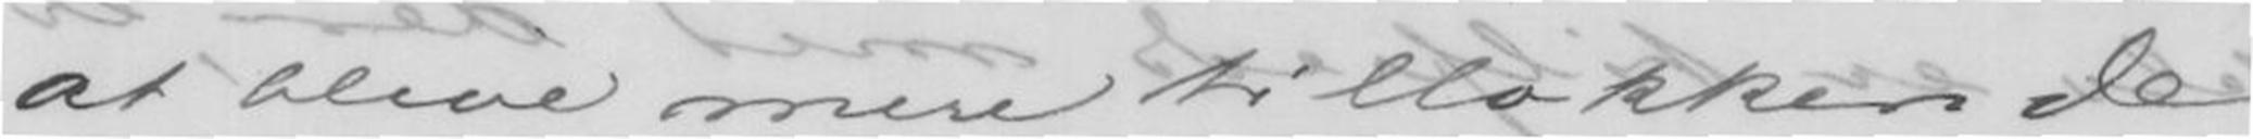at blive mere tillokkende
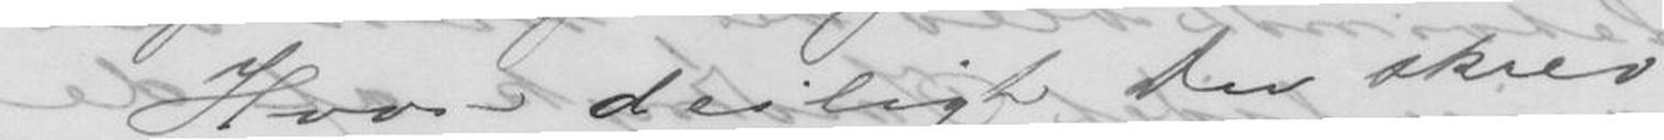Hvor deiligt Du skrev
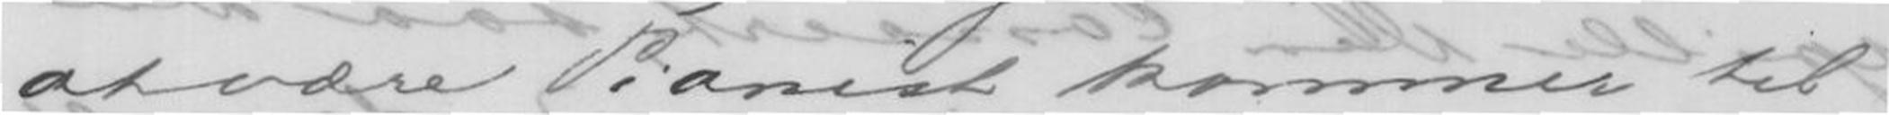at være Pianist kommer til
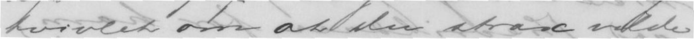tvivlet om at Du strax vilde
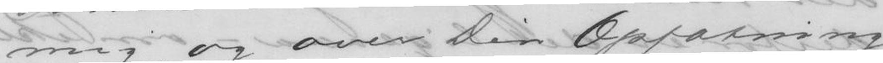mig og over Din Opfatning
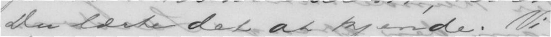Du lærte det at kjende. Vi
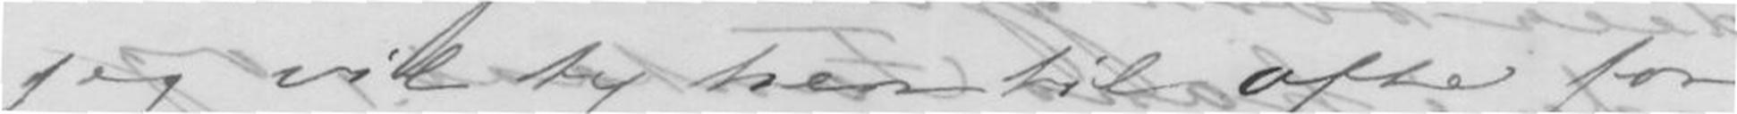jeg vil ty hen til ofte for
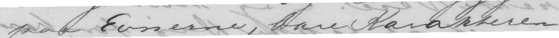paa Evnerne, bare Karakteren
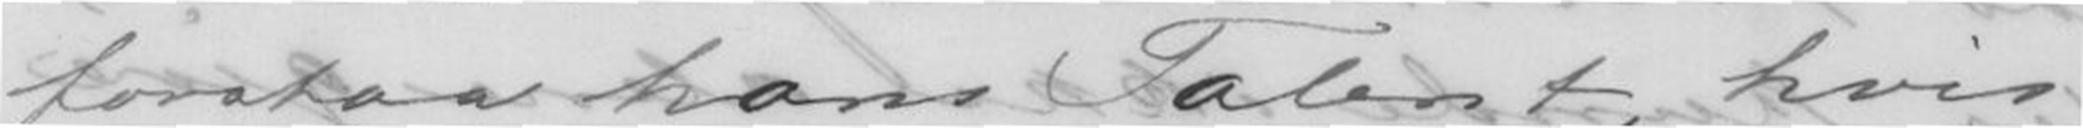forstaa hans Talent, hvis
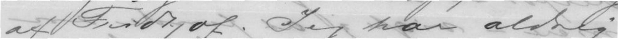af Fridtjof. Jeg har aldrig
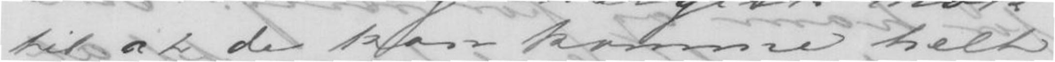til at de kan komme helt
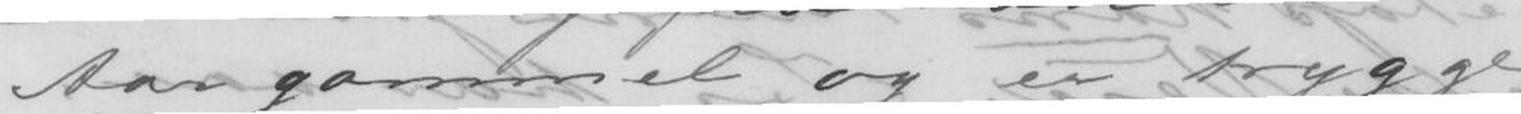Aar gammel og er trygge
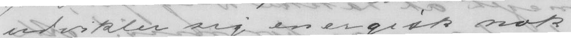udvikler sig energisk nok
